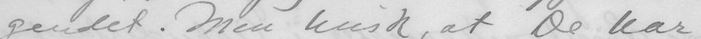gendet. Men husk, at De har
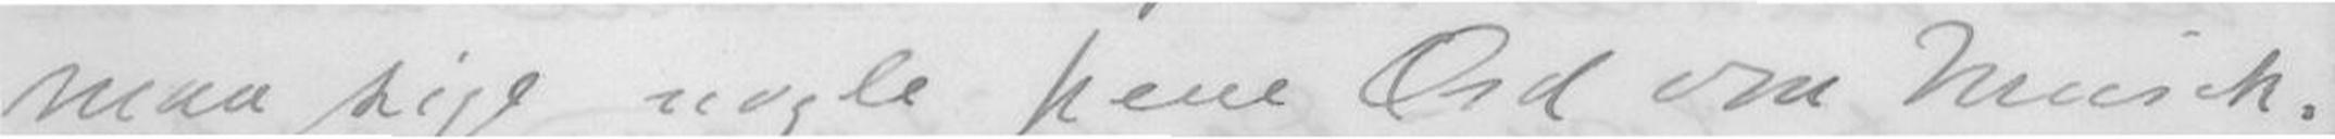maa sige nogle pene Ord om Musik.
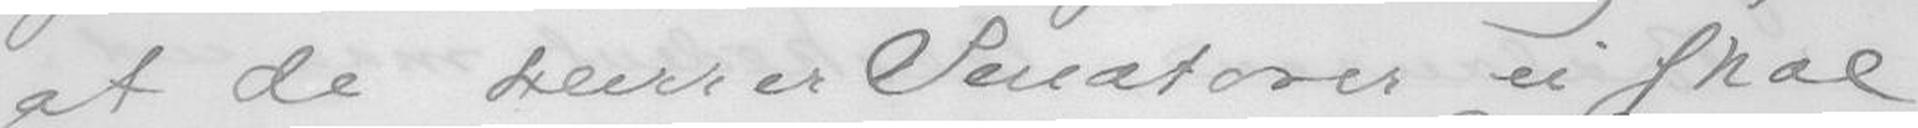at de Herrer Senatorer ei skal
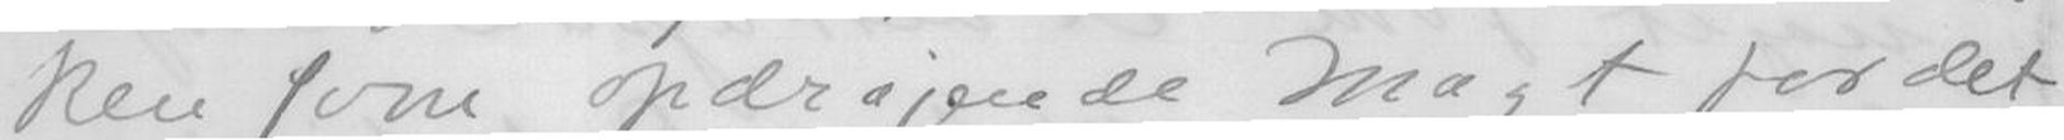Dem som opdragende Magt for det
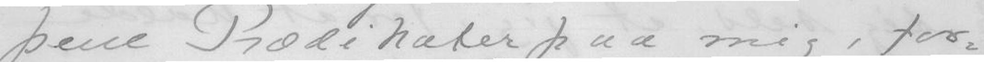pene Prædikater paa mig, for-
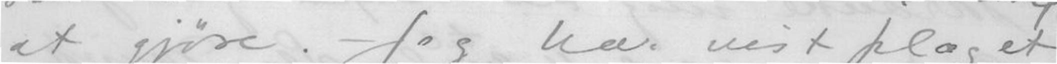at gjøre.- Jeg har vist plaget
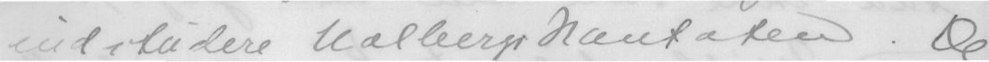indstudere Holberg kantaten. De
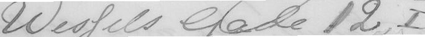Wessels Gade 12, I
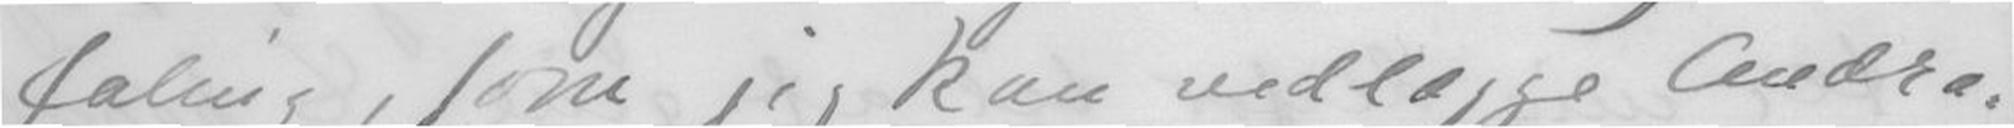faling, som jeg kan vedlægge Andra-
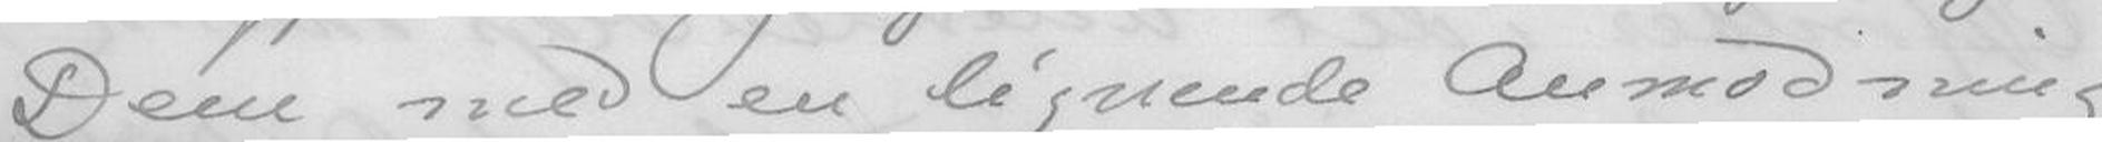Dem med en lignende Anmodning
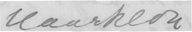J. Haarklau.
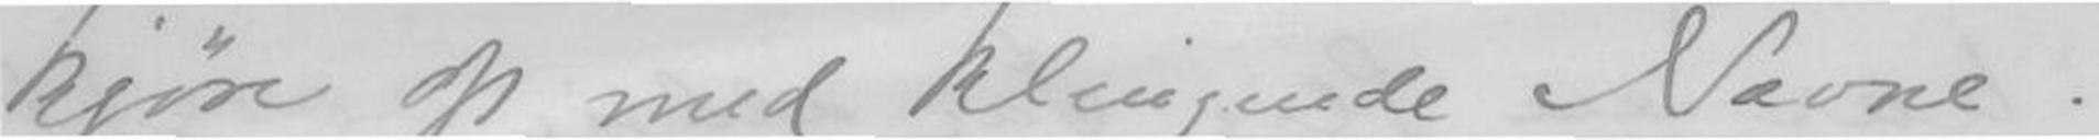kjøre op med klingende Navne.
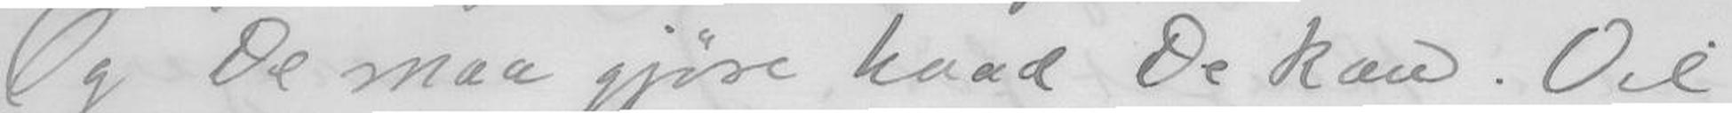Og De maa gjøre hvad De kan. Vil
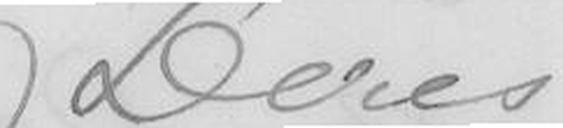Deres
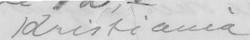Kristiania
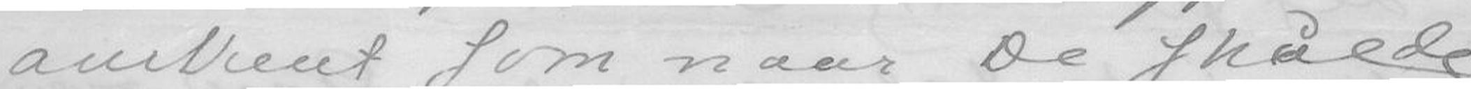omtrent som naar De skulde
